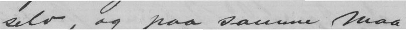selv, og paa samme Maa-
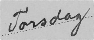Torsdag
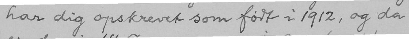har dig opskrevet som født i 1912, og da
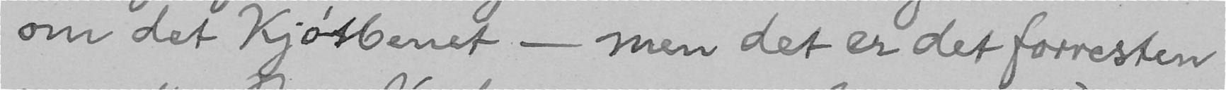om det Kjøtbenet - men det er det forresten
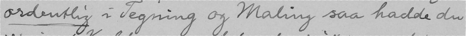ordentlig i Tegning og Maling saa hadde du
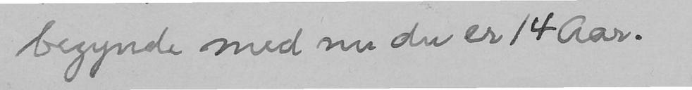begynde med nu du er 14 Aar.
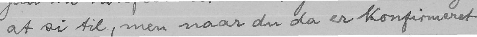at si til, men naar du da er konfirmeret
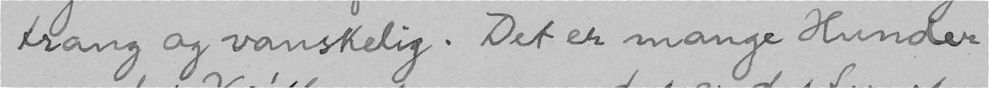trang og vanskelig. Det er mange Hunder
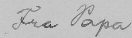Fra Papa
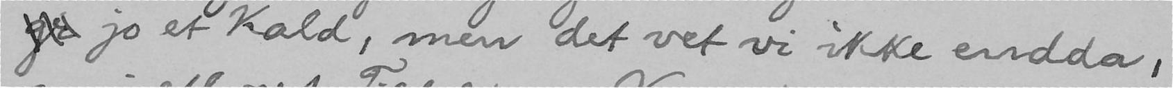ge jo et Kald, men det vet vi ikke endda,
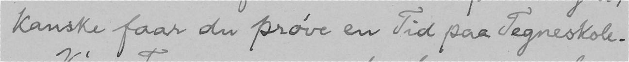kanske faar du prøve en Tid paa Tegneskole.
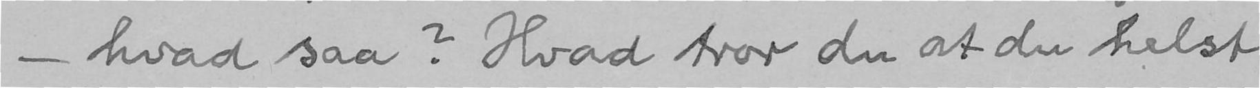- hvad saa? Hvad tror du at du helst
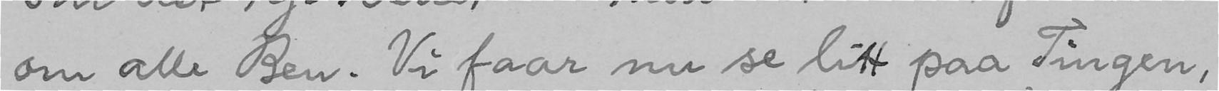om alle Ben. Vi faar nu se litt paa Tingen,
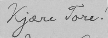Kjære Tore!
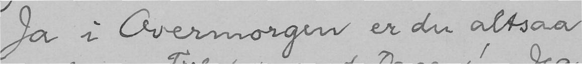Ja i Overmorgen er du altsaa
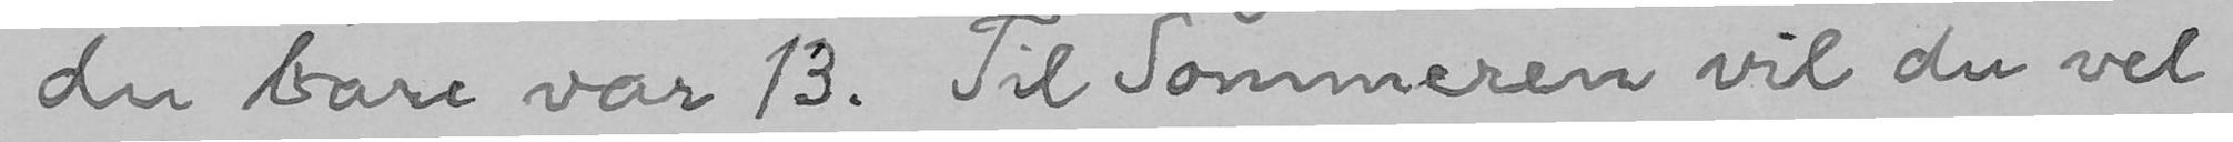du bare var 13. Til Sommeren vil du vel
